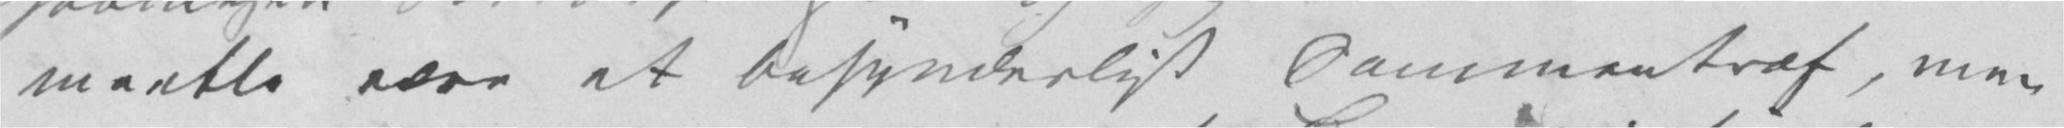maatte være et besynderligt Sammentræf, men
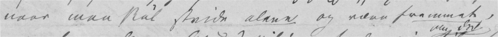naar man skal stride alene og være fremmet,
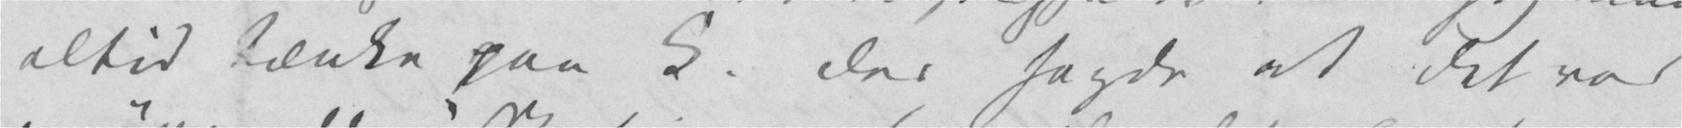altid tænke paa C. der sagde at det var
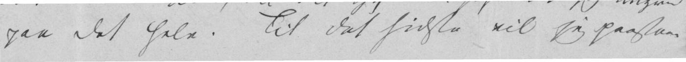paa det hele. Til det sidste vil jeg paastaae
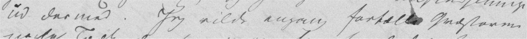ud dermed. Jeg vilde engang fortælle Qvæstoren
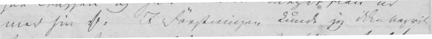med sin [Ort]. I Førstningen kunde jeg ikke begribe
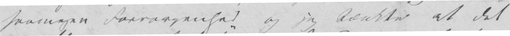saamegen Forvorpenhed og jeg tænkte at det
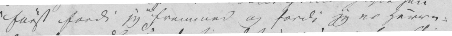«først fordi jeg er fremmed og fordi jeg er Herre-
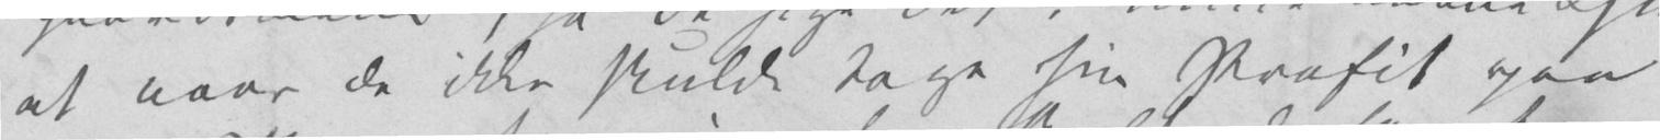at naar de ikke skulde tage sin Profit paa
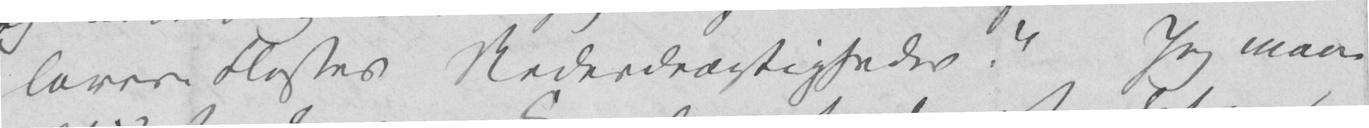lavere Klasses Nederdrægtigheder.» Jeg maae
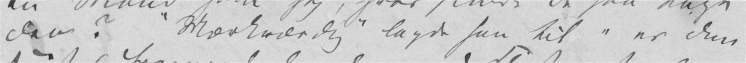den? «Mærkværdig» lagde han til «er den
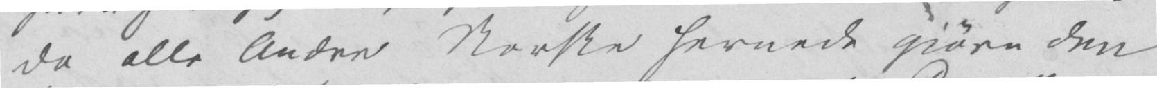da alle Andre Norske hernede gjøre den
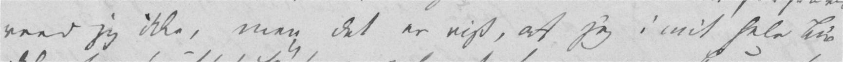veed jeg ikke, men det er vist, at jeg i mit hele Liv
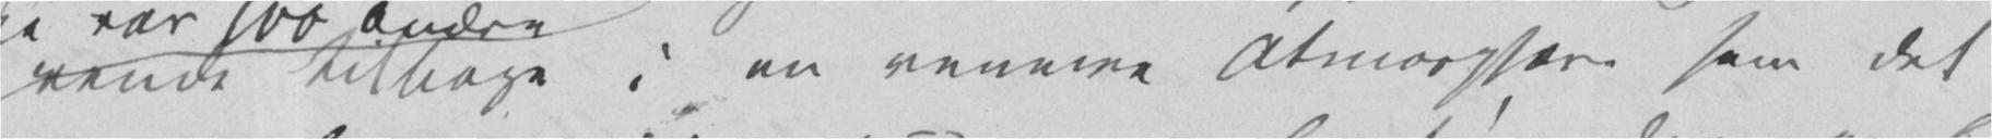vende tilbage i en renere Atmosphære som det
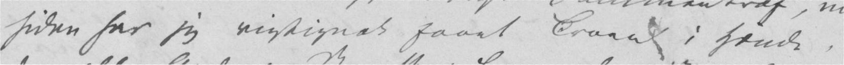siden har jeg rigtignok faaet Troen i Hænde,
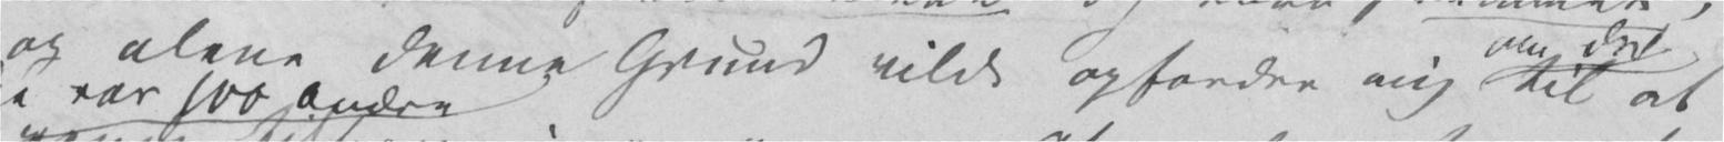og alene denne Grund vilde opfordre mig om der til at
